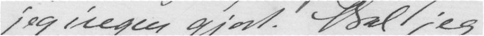jeg ingen gjort. Skal jeg
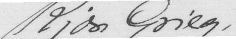Kjære Grieg,
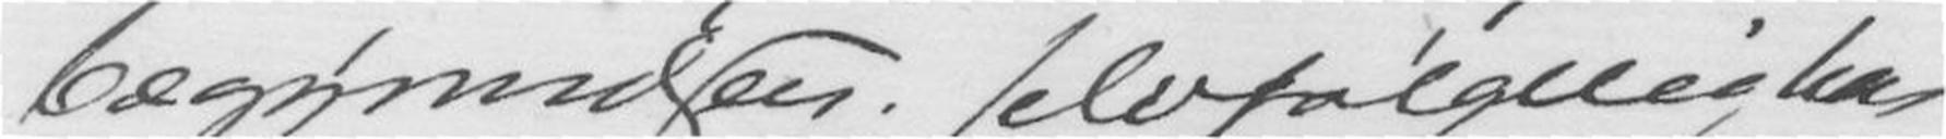begyndelsen; selvfølgelig har
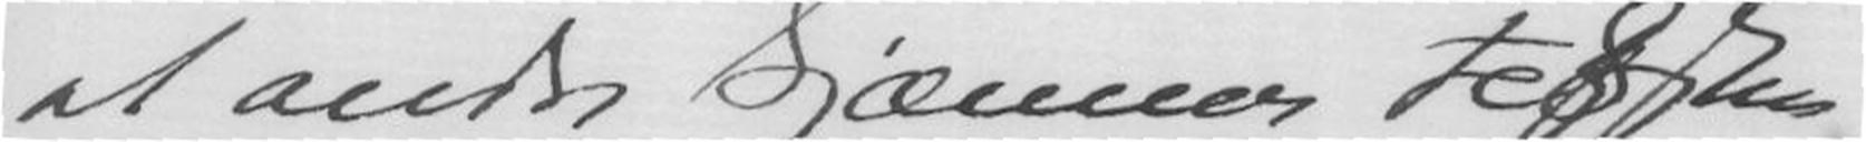at andre kjænner teksten.
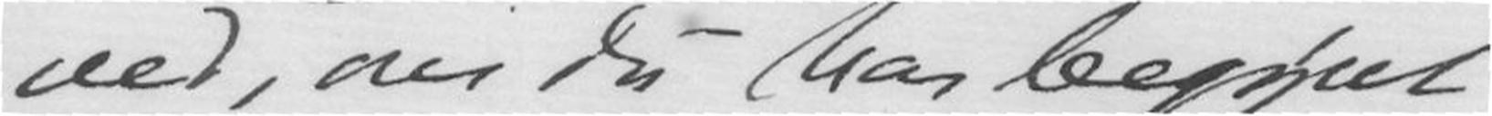ved, om du har begynt
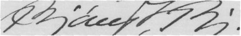Bjørnst, Bj.
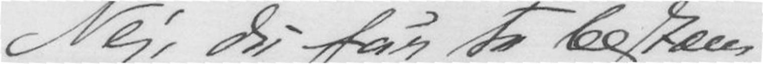Nej, du får ta bestæm-
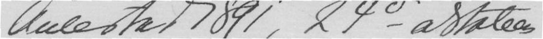Aulestad 1891, 24deoktober
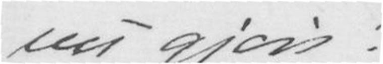nu gjøre?
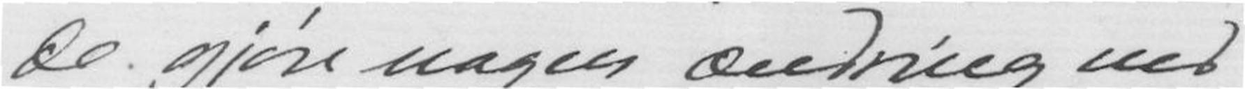de gjøre nogen ændring ved
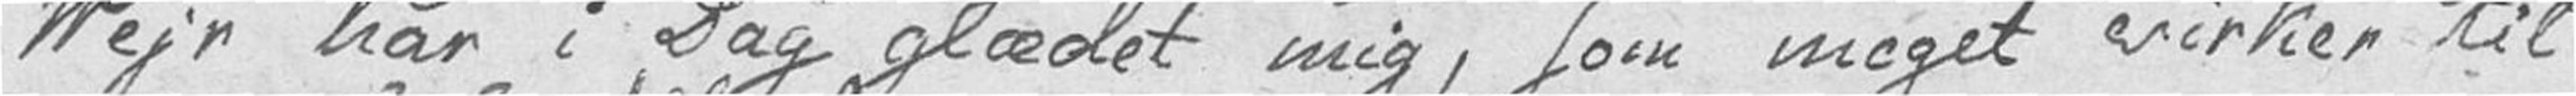Vejr har i Dag glædet mig, som meget virker til
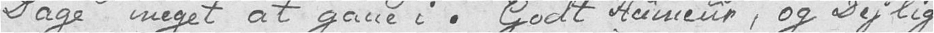Dage meget at gaae i. Godt Humeur, og Dejlig
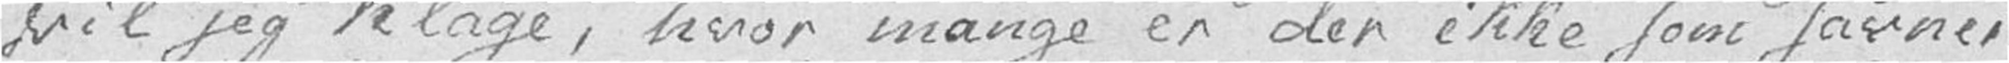vil jeg Klage, hvor mange er der ikke som savner
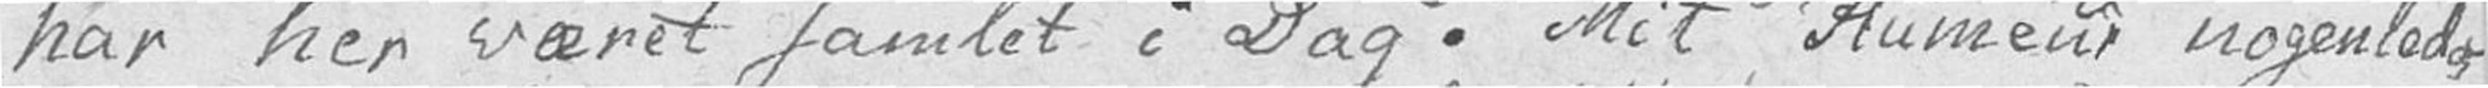har her været samlet i Dag. Mit Humeur nogenledes
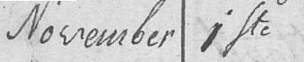November 1ste
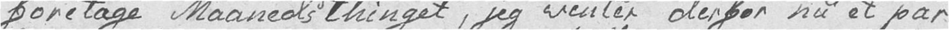foretage Maanedsthinget, jeg venter derfor nu et par
